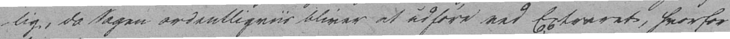lig, da Sagen ordentligviis bliver at udføre ved Extraret, hvorfor
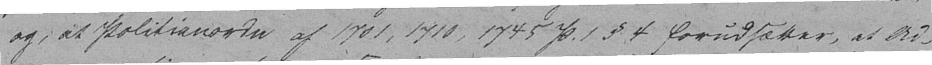og, at Politianordn af 1701, 1710, 1745 P. 1 § 4 forudsætter, at Ad-
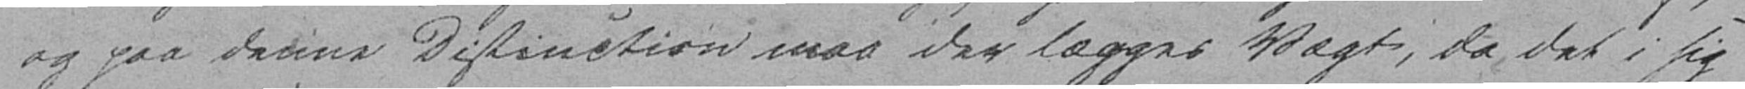og paa denne Distinction maa der lægges Vægt, da det i sig
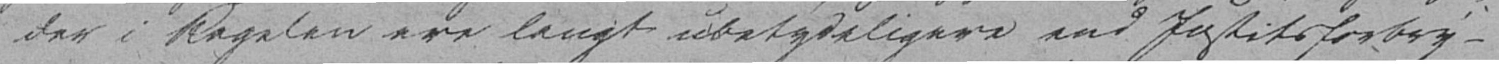der i Regelen ere langt ubetydeligere end Justitsforbry-
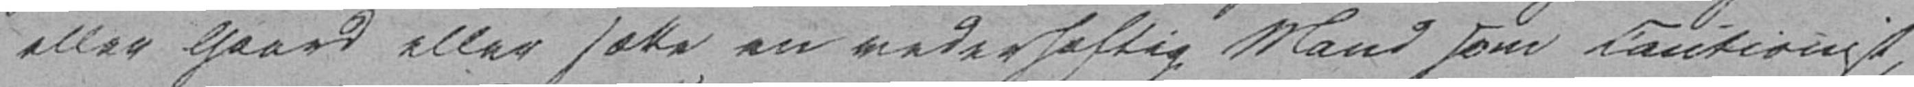eller Gaard eller sætte en vederhæftig Mand som Cautionist,
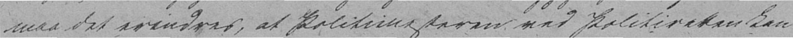maa det erindres, at Politimesteren ved Politiretten kan
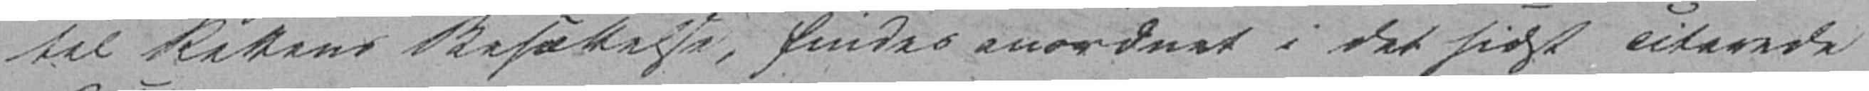til Rettens Besættelse, findes anordnet i det sidst citerede
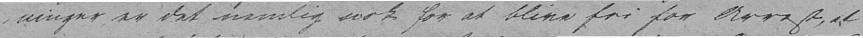ninger er det nemlig nok for at blive fri for Arrest, at
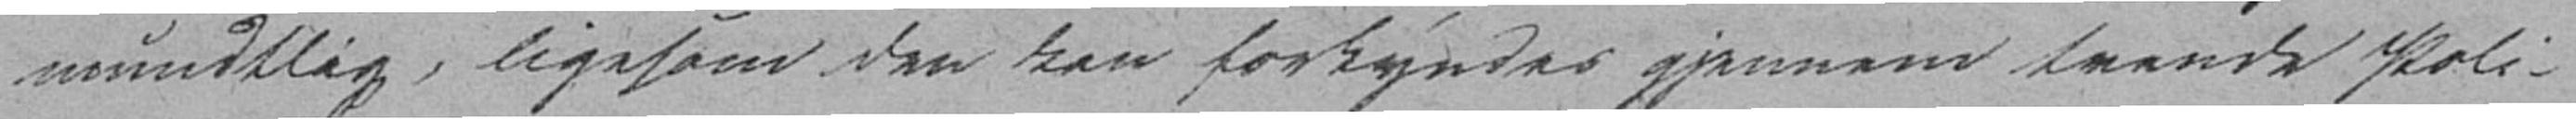mundtlig, ligesom den kan forkyndes gjennem tvende Poli-
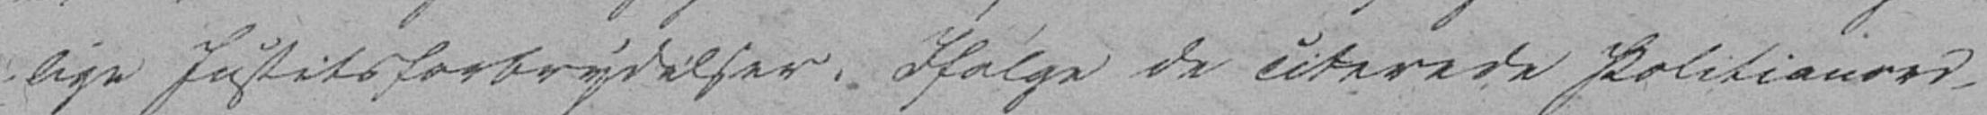lige Justitsforbrydelser. Ifølge de citerede Politianord-
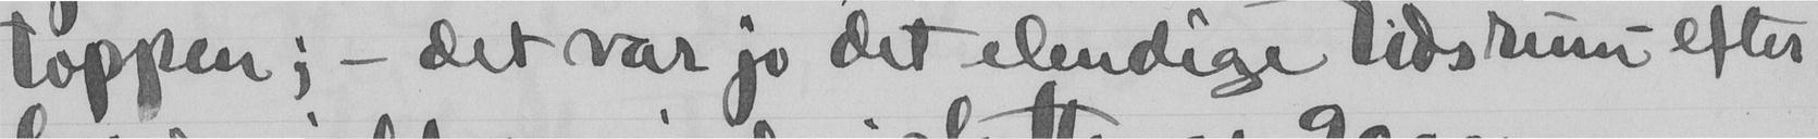toppen; - det var jo det elendige tidsrum efter
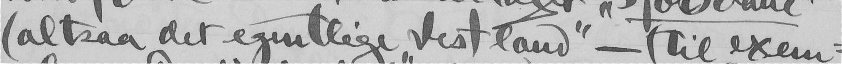(altsaa det egentlige "Vestland" - (til exem-
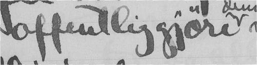offentliggjøre
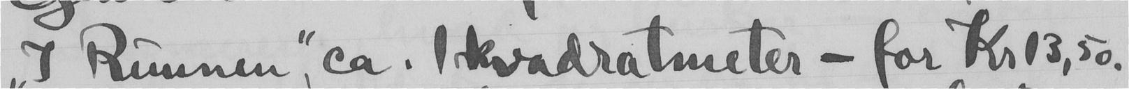"I Runnen", ca. 1 Kvadratmeter - for Kr 13,50.
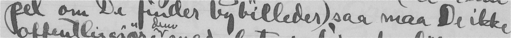pel om De finder bybilleder) saa maa De ikke
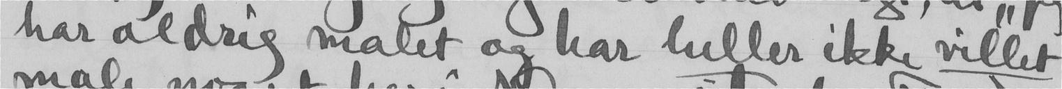har aldrig malet og har heller ikke villet
